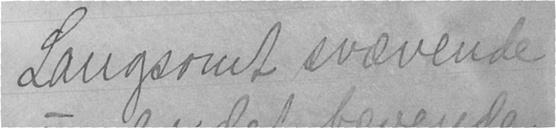Langsomt svævende
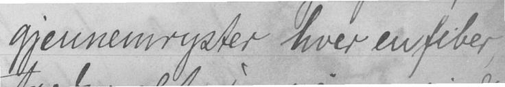gjennemryster hver en fiber,
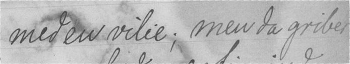med en vilie; men da griber
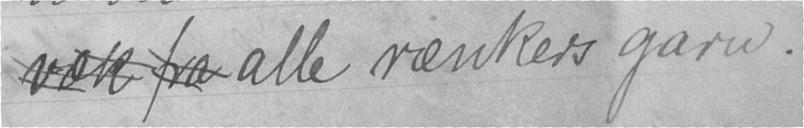væk fra alle rænkers garn.
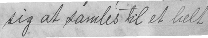sig at samles til et helt
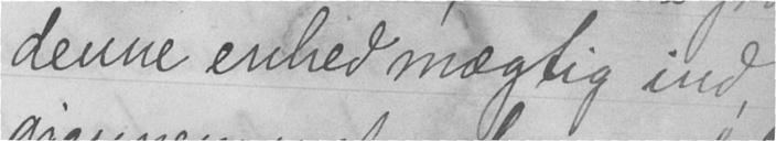denne enhed mægtig ind,
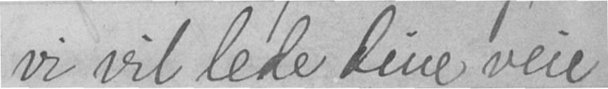vi vil lede dine veie
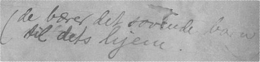(de bærer det sovende barn til dets hjem.)
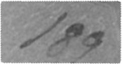189.
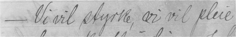- Vi vil styrke, vi vil pleie
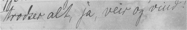trødser alt, ja, veir og vind!
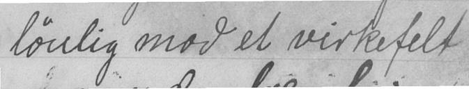lønlig mod et virkefelt
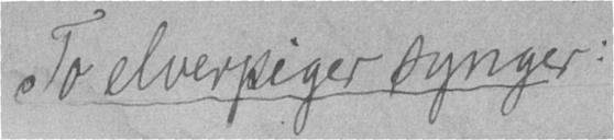To elverpiger synger:
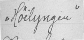"Høilyngen"
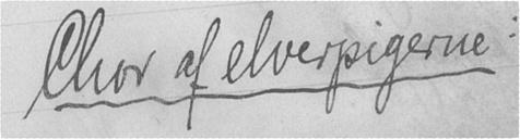Chor af elverpigerne:
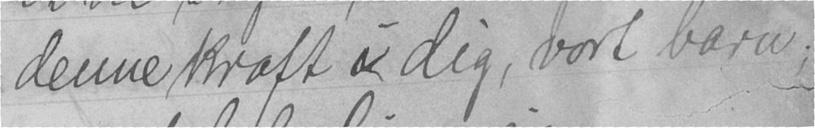denne kraft i dig, vort barn;
